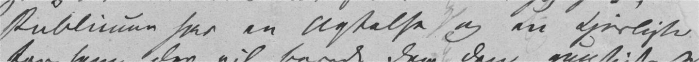Publicum har en Agtelse og en Kjerlighed
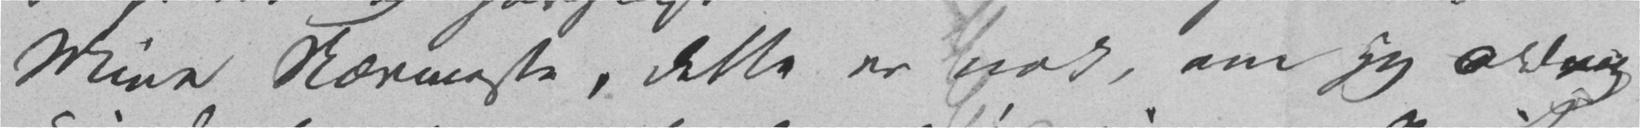Mine Nærmeste, dette er nok, om jeg aldrig
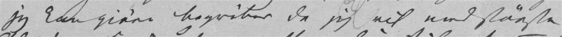jeg kan gjøre begriber De jeg vil med største
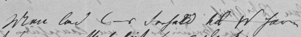Men lad C-s Forhold til W have
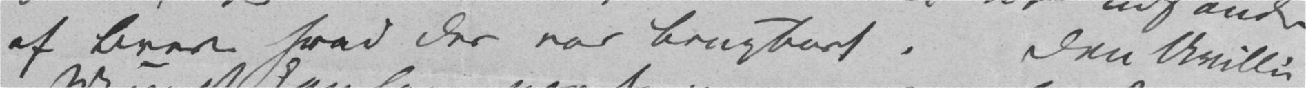af Breve hvad der var brugbart. Den Uvillie
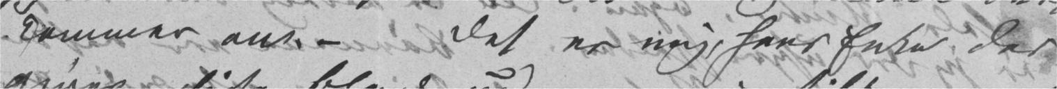kommer an. – Det er mig, hans Enke der
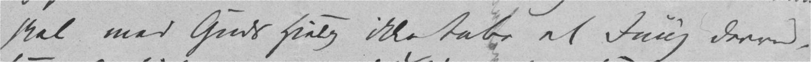skal med Guds Hjelp ikke tabe et Fnug derved.
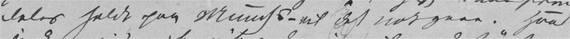deles holde paa Munchs – vil det nok gaae. Hvad
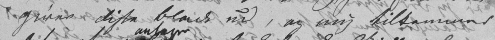giver disse Blade ud, og mig tilkommer
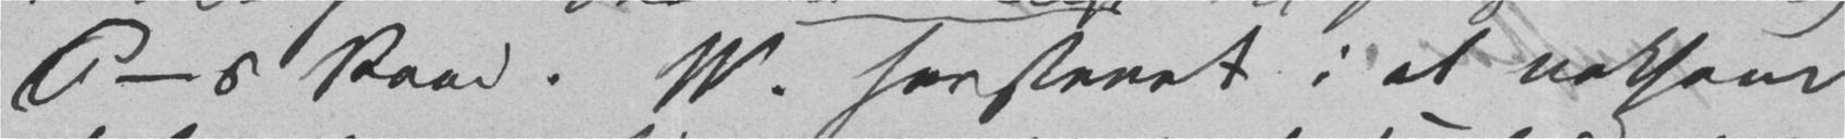C-s Raad. W. har staaet i et noksom
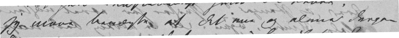jeg maae benægte at det ene og alene derpaa
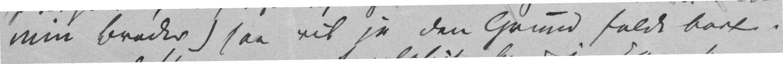min Broder) saa vil jo den Grund falde bort.
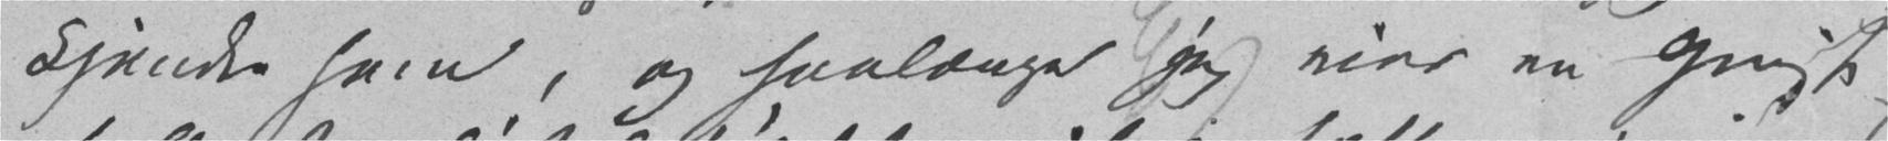kjendte ham, og saalænge jeg eier en Gnist
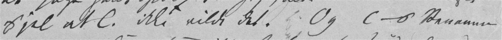Sjel at C. ikke vilde det. Og C-s Renomme
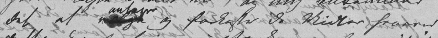det at vælge antage og forkaste de Midler hvorved
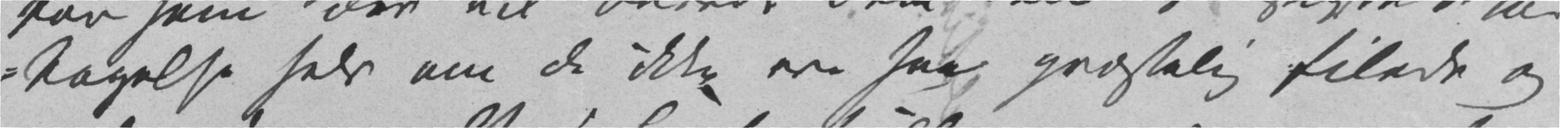tagelse selv om de ikke ere saa græsselig filede og
